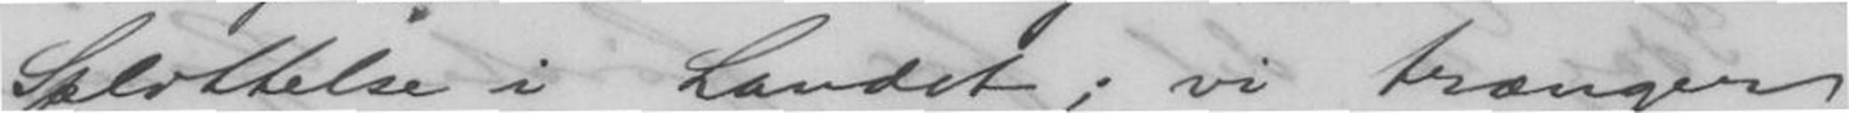Splittelse i Landet; vi trænger
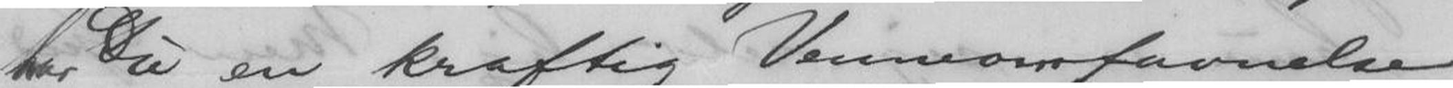har Du en kraftig Venneomfavnelse
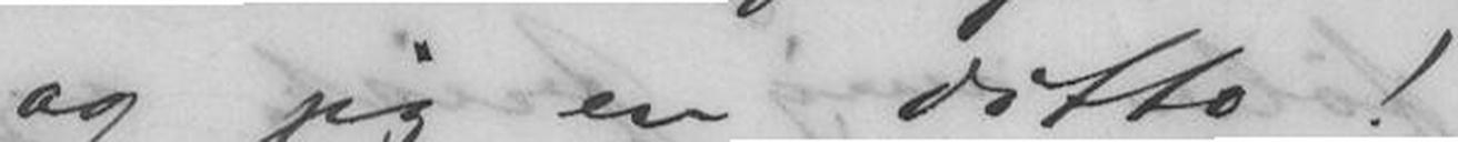og jeg en ditto!
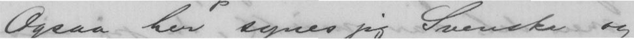Ogsaa her synes jeg Svenske og
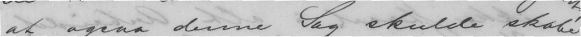at ogsaa denne Sag skulde skabe
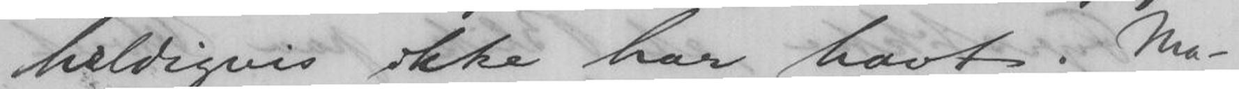heldigvis ikke har havt. Ma-
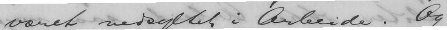været nedsyltet i Arbeide. Og
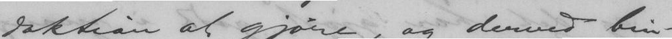daktion at gjøre, og dermed bin-
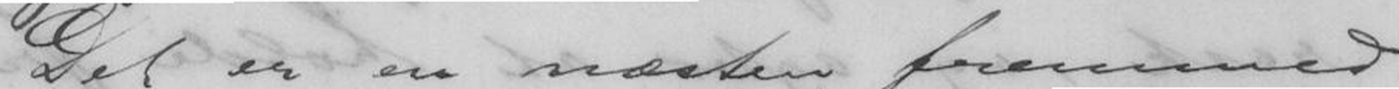Det er en næsten fremmed
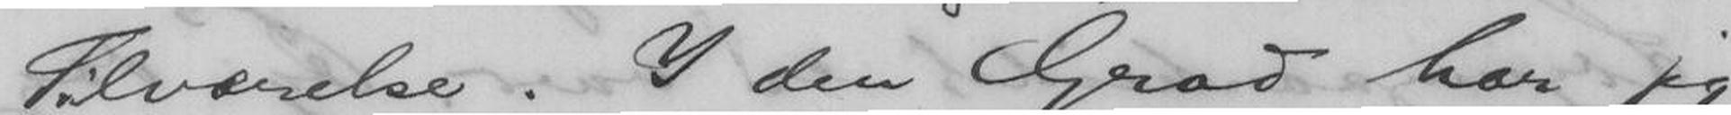Tilværelse. I den Grad har jeg
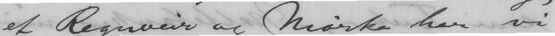et Regnveir og Mørke har vi
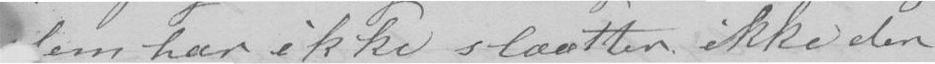lem har ikke slaatten ikke den
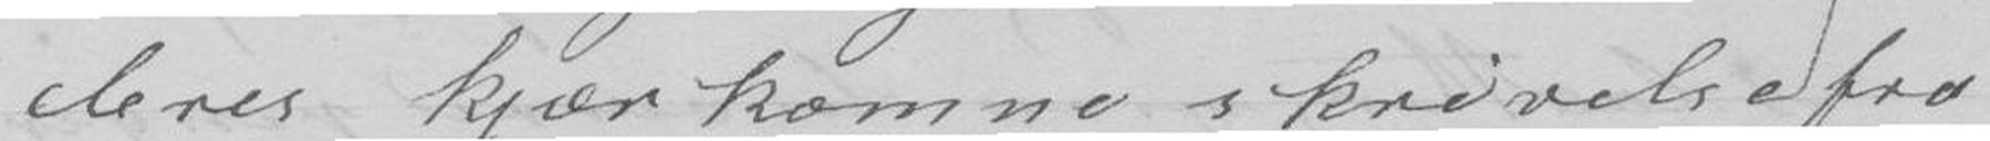deres kjærkomne skrivelse fra
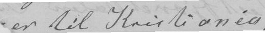er til Kristiania
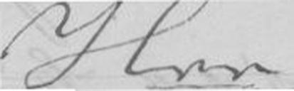Hrr
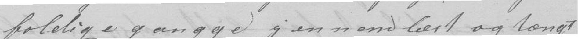foldige gangge jennemlæst og tængt
